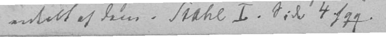enkelt af dem. Stahl I. Side 4 197.
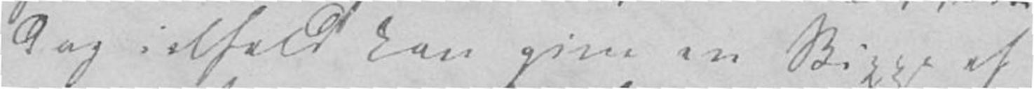dog ialfald kan give en Skizze af
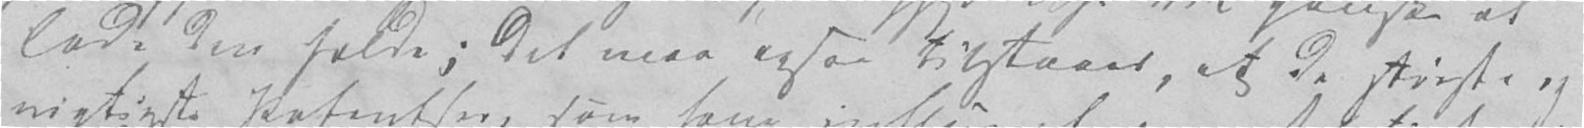lade den falde; det maa ogsaa tilstaaes, at de største og
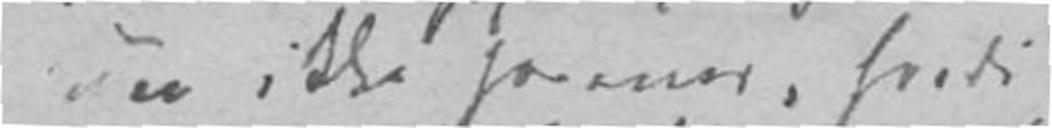nu ikke forenes, fordi
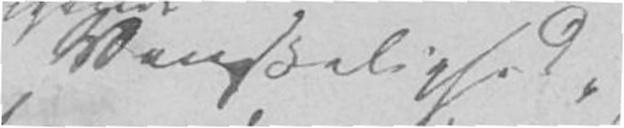Vanskelighed.
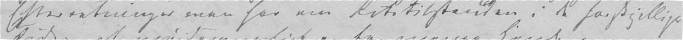Efterretninger man har om Retstilstanden i de forskjellige
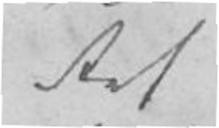Ret
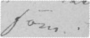som i
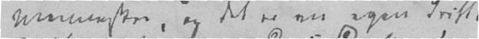Mennesker, og det er en egen Drift
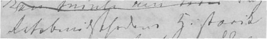Retsbevidsthedens Historie
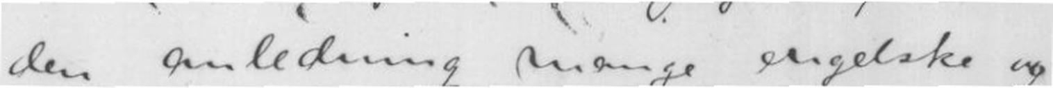den anledning mange engelske og
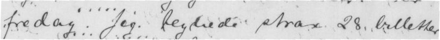fredag. Jeg tegnede strax 28. billetter
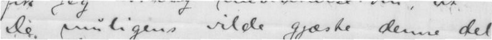De muligens vilde gjæste denne del
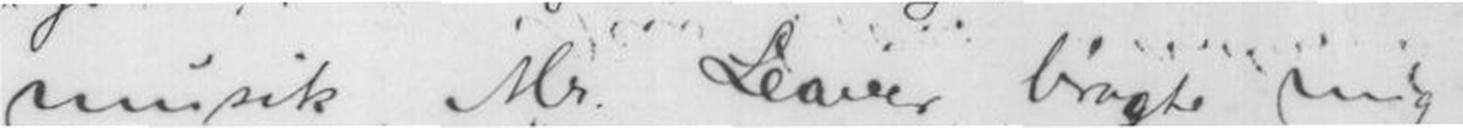musik. Mr. Leaver bragte mig
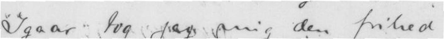Igaar tog jeg mig den frihed
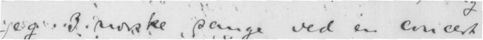jeg 3 norske sange ved en concert
